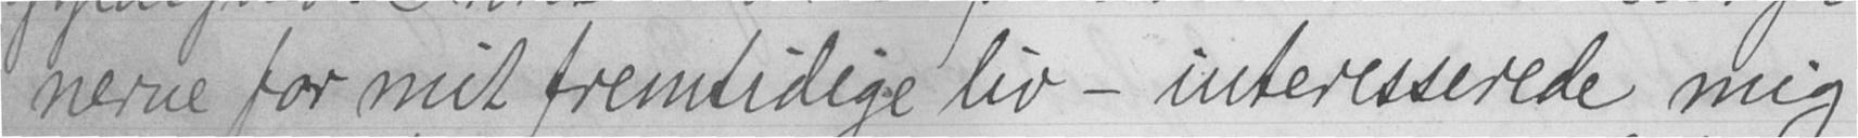nerne for mit fremtidige liv - interesserede mig
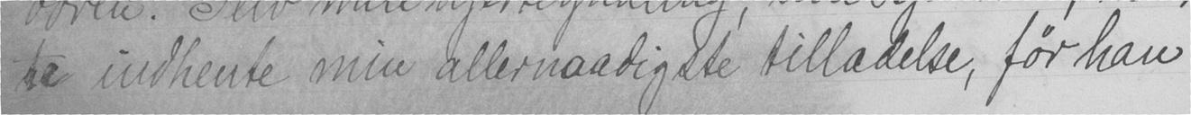te indhente min allernaadigste tilladelse, før han
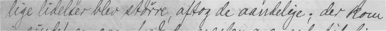lige lidelser blev større, aftog de aandelige; der kom
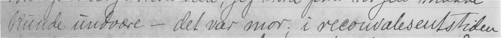kunde undvære - det var mor, i recouvalesentstiden
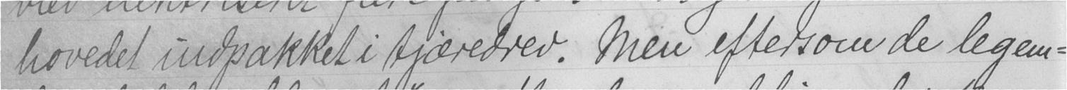hovedet indpakket i tjæredrev. Men eftersom de legem-
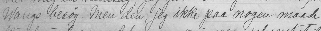Wangs besøg. Men den, jeg ikke paa nogen maade
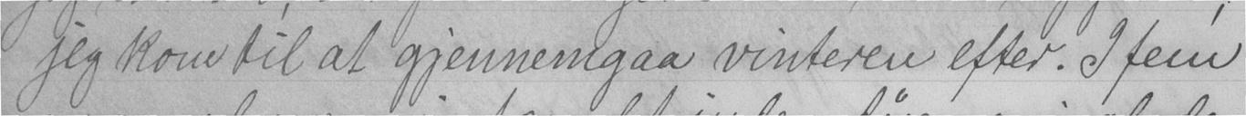jeg kom til at gjennemgaa vinteren efter. I fem
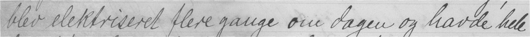blev elektriseret flere gange om dagen og havde hele
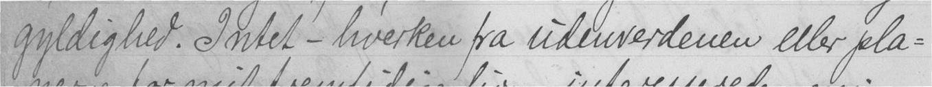gyldighed. Intet - hverken fra udenverdenen eller pla-
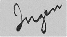Ingen
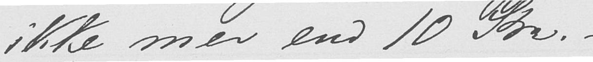ikke mer end 10 Kr. -
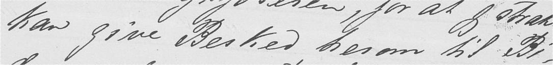kan give Besked herom til Bi-
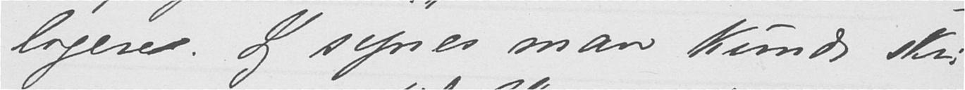ligere. Jeg synes man kunde skri-
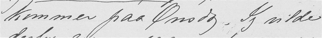kommer paa Onsdag. Jeg vilde
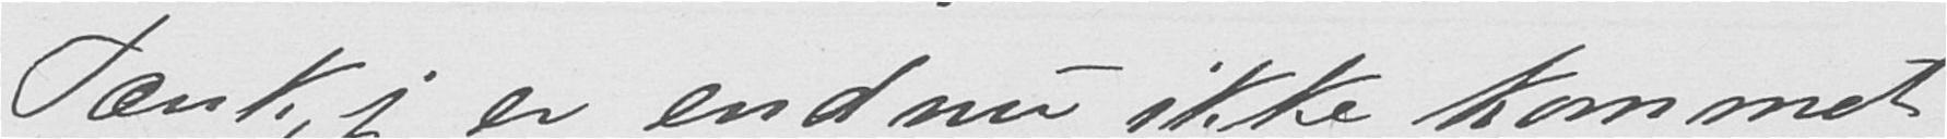Tænk jeg er endnu ikke kommet
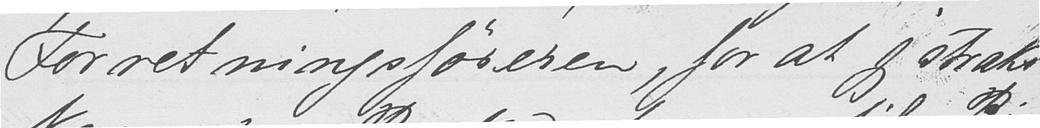Forretningsføreren, for at jeg straks
